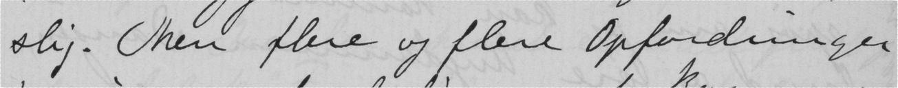slig. Men flere og flere Opfordringer
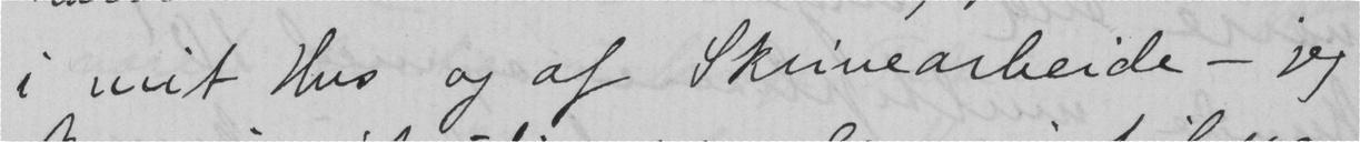i mit Hus og af Skrivearbeide - jeg
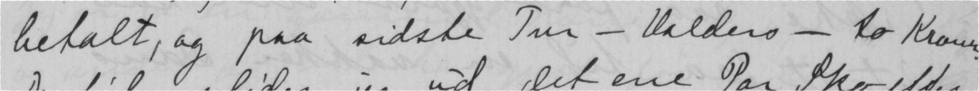betalt, og paa sidste Tur - Valders - to Kroner.
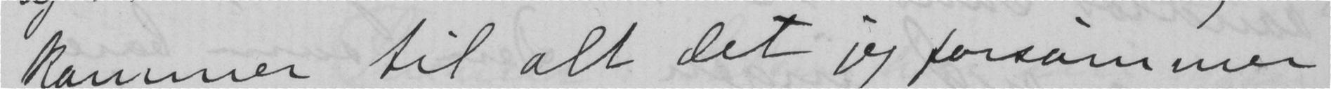kommer til alt det jeg forsømmer
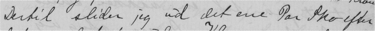Dertil slider jeg ud det ene Par Sko efter
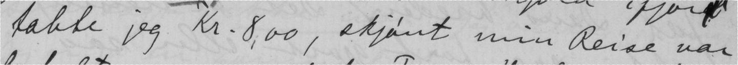tabte jeg Kr. 8,00, skjønt min Reise var
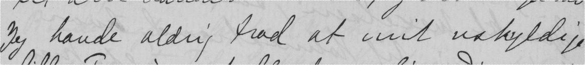Jeg havde aldrig trod at mit uskyldige
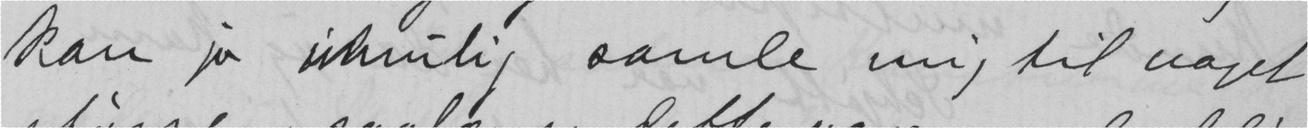kan jo umulig samle mig til noget
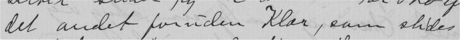det andet foruden Klær, som slides
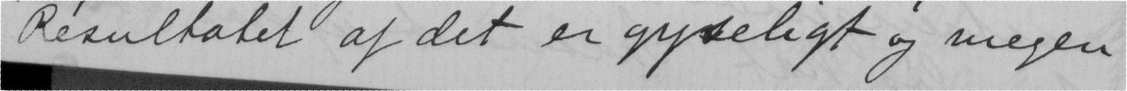Resultatet af det er gyseligt og megen
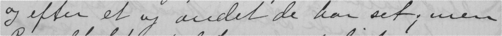og efter et og andet de har set; men
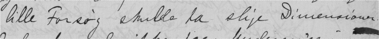lille Forsøg skulde ta slige Dimensioner.
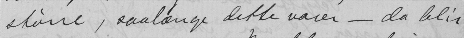større, saalænge dette varer - da blir
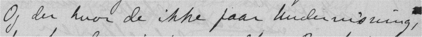Og der hvor de ikke faar undervisning,
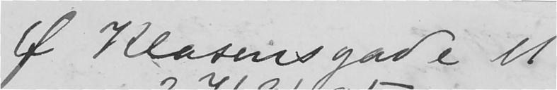Ø Klasensgade 11
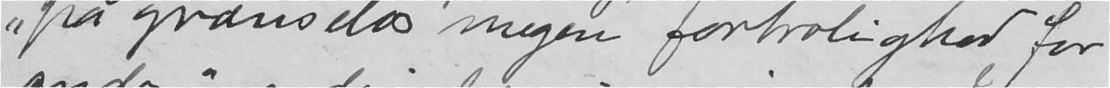"på grænseløs megen fortrolighed for
